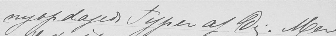nyopdagede Typer af Dig. Mer
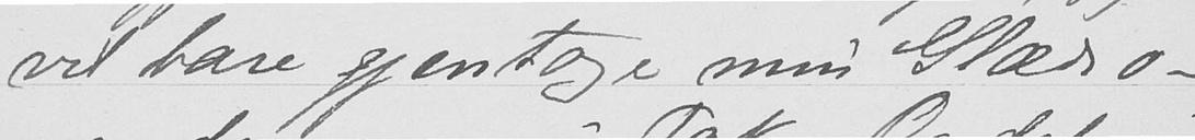vil bare gjentage min Glæde o-
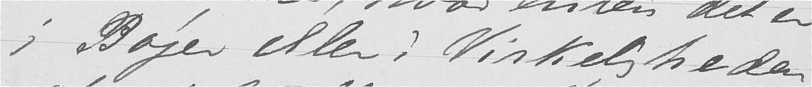i Bøger eller i Virkeligheden,
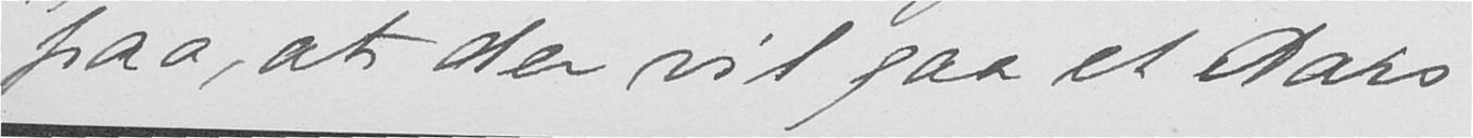paa, at der vil gaa et Aars
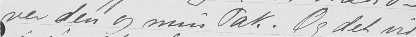ver den og min Tak. Og det vil
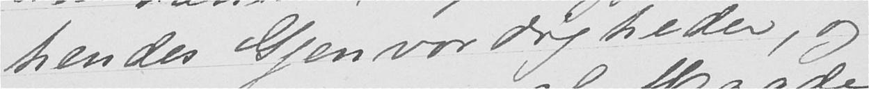hendes Gjenvordigheder, og
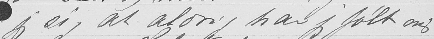jeg si, at aldrig har jeg følt mig
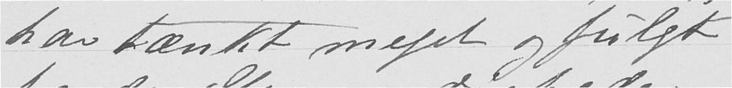har tænkt meget og fulgt
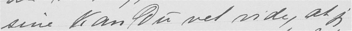sine kan Du vel vide, at jeg
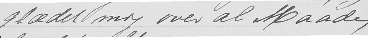glædet mig over al Maade,
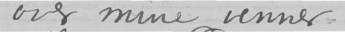over mine venner.
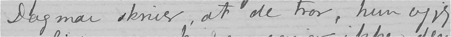Dagmar skriver, at de tror, hun og jeg
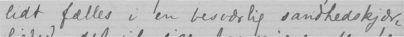lidt fælles i en besværlig sandhedskjær-
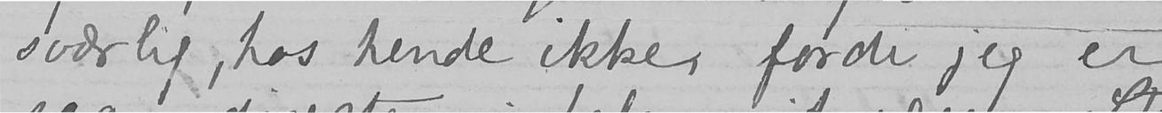sværlig, hos hende ikke, fordi jeg er
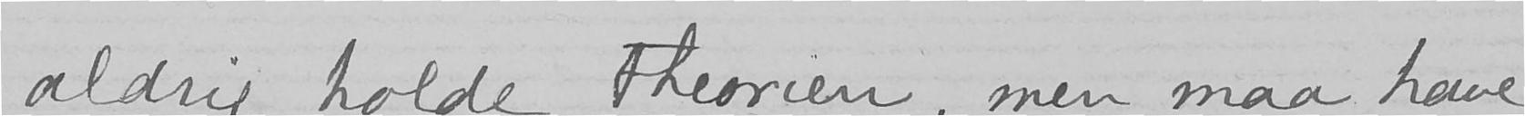aldrig holde theorien, men maa have
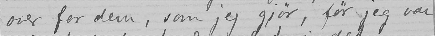over for dem, som jeg gjør, før jeg var
